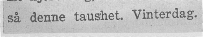så denne taushet. Vinterdag.
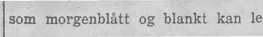som morgenblått og blankt kan le
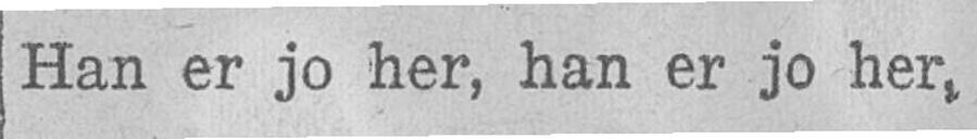Han er jo her, han er jo her,
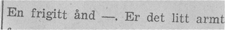En frigitt ånd —. Er det litt armt
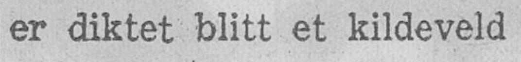er diktet blitt et kildeveld
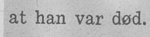at han var død.
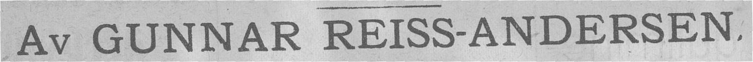Av GUNNAR REISS-ANDERSEN.
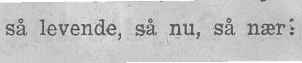så levende, så nu, så nær:
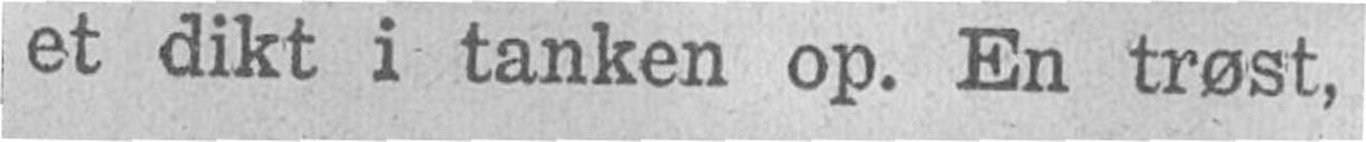et dikt i tanken op. En trøst,
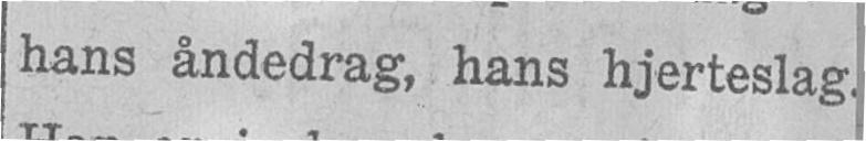hans åndedrag, hans hjerteslag
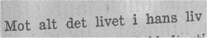Mot alt det livet i hans liv
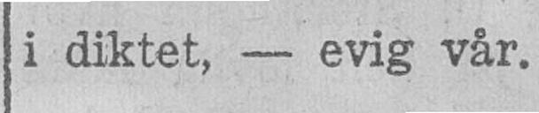i diktet, — evig vår.
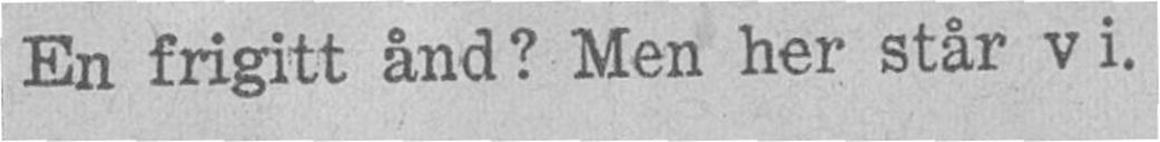En frigitt ånd? Men her står vi.
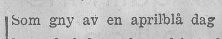Som gny av en aprilblå dag
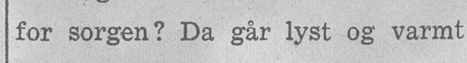for sorgen? Da går lyst og varmt
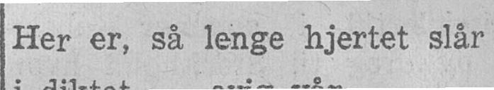Her er, så lenge hjertet slår
